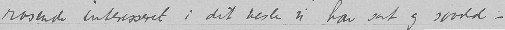rasende interesseret i det vesle vi har vært sat og saadd -
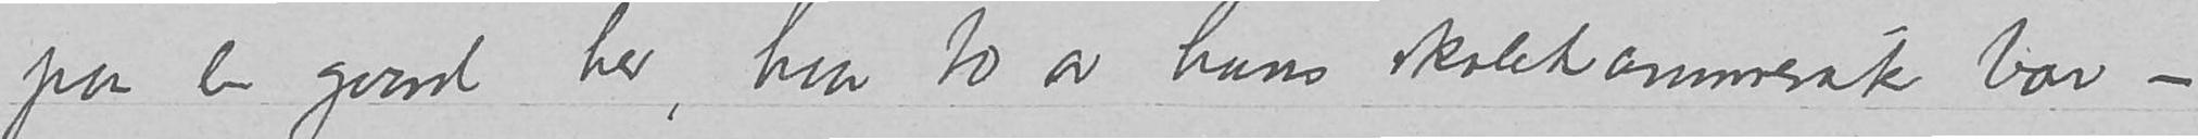paa en gaard her, hvor to av hans skolekammerater bor -
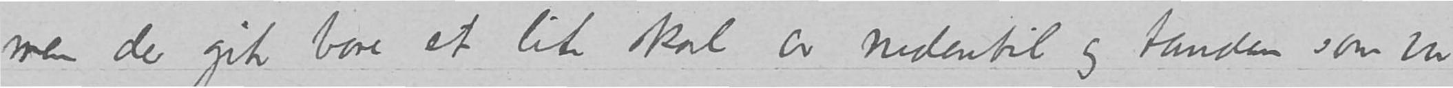men der gik bare et lite skal av nedentil og tanden som var
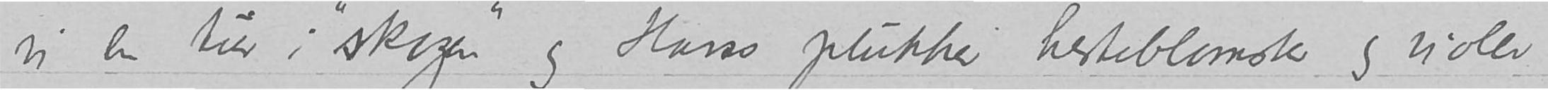vi en tur i «skogen» og Hans plukker hesteblomster og violer
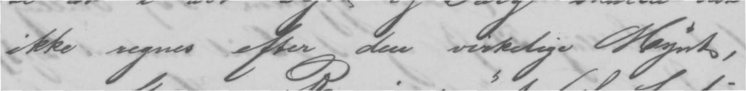ikke regnes efter den virkelige Mynt,
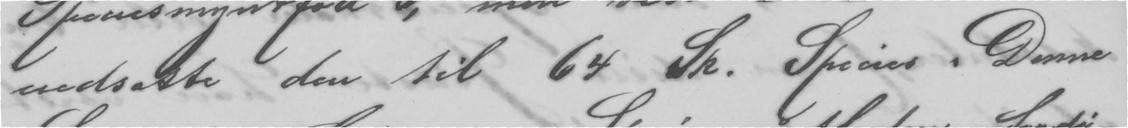nedsatte den til 64 Sk. Species. Denne
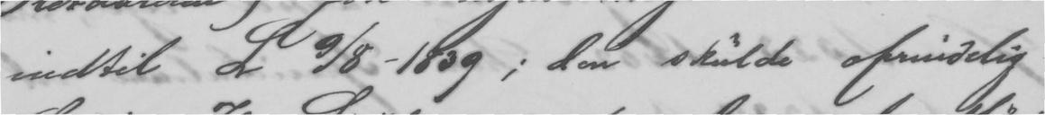indtil I 9/8 - 1839; den skulde oprindelig
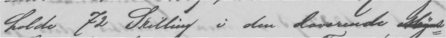holde 72 Skilling i den daværende Mynt-
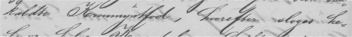kaldte Kronsmyntfod, hvorefter sloges he-
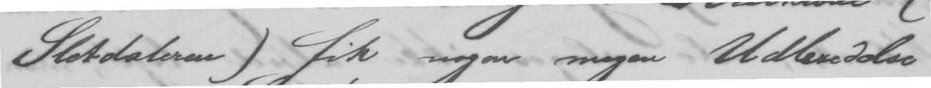Sletdaleren) fik nogen megen Udbreddelse
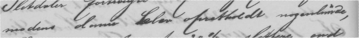modens dom heler opretholdt nogenlunde,
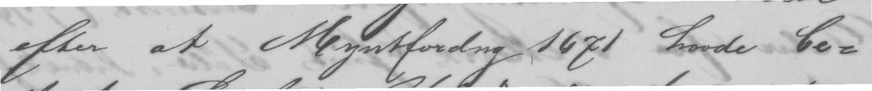efter at Myntfordng 1671 havde be-
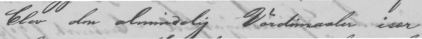blev den almindelig Værdimaaler især
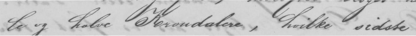le og halve Krondalere, hvilke sidste
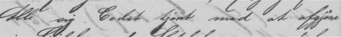Alle sig bedst tjent med at afgjøre
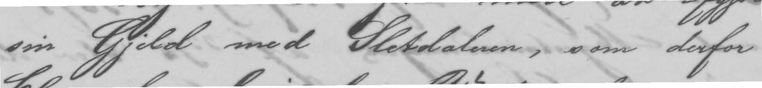sin Gjeld med Sletdaleren, som derfor
